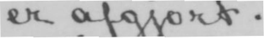er afgjort.
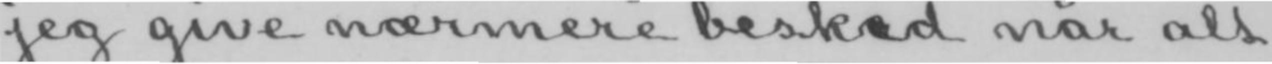jeg give nærmere besked når alt
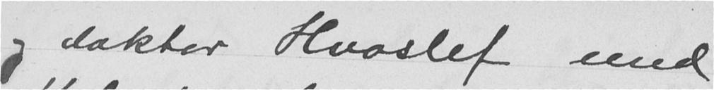og doktor Hvoslef med
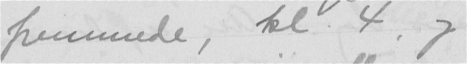fremmede, kl. 4, og
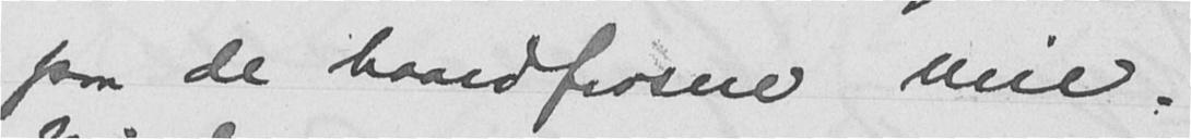paa de haardfrosne veie.
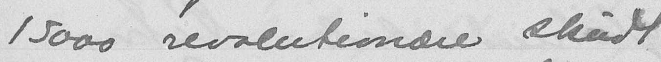15000 revolutionære skudt
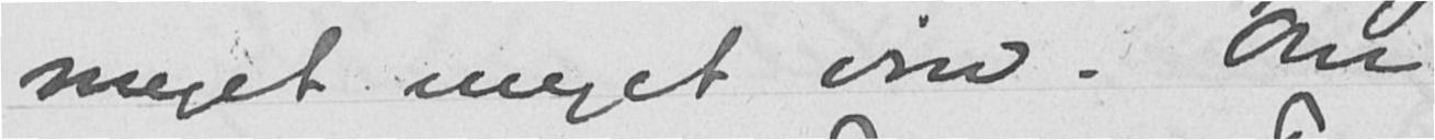meget meget vin. Om
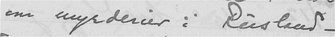om myrderier i Rusland -
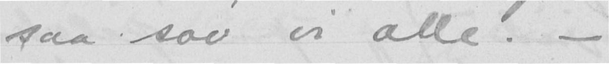saa sov vi alle. -
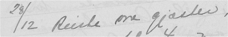28/12 Reiste vore gjæster,
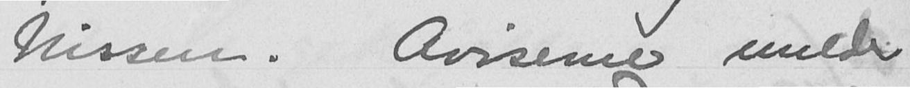Nissen. Aviserne melder
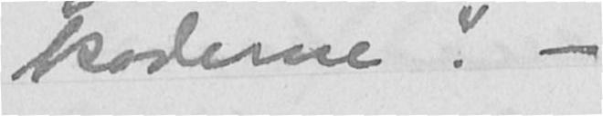kaderne." -
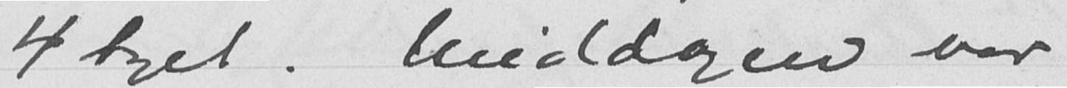4 toget. Middagen var
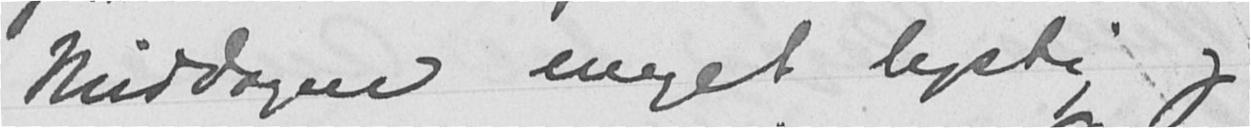Middagen meget lystig, og
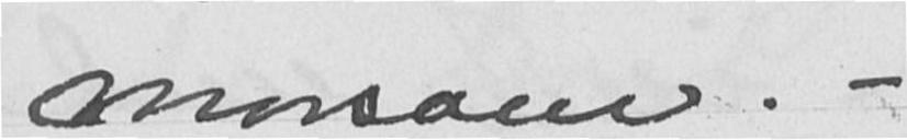morsom. -
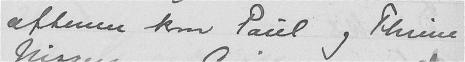aftenen kom Paul og Thrine
